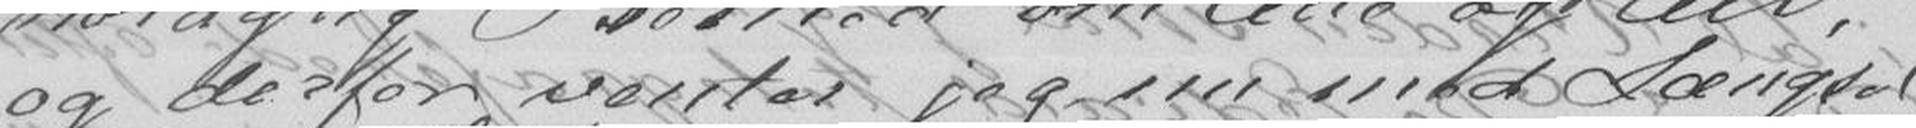og derfor ventes jeg nu med Længsel
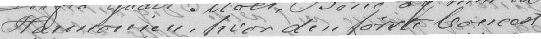Harmonien, hvor den første Concert
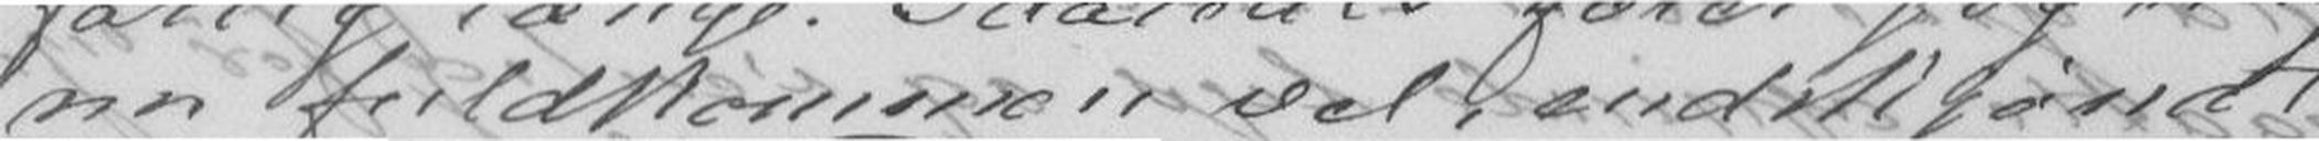nu fuldkommen vel, endskjøndt
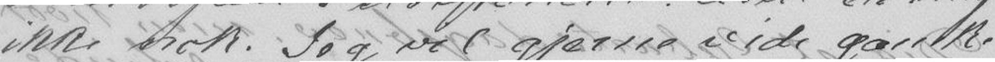ikke nok. Jeg vil gjerne vide ganske
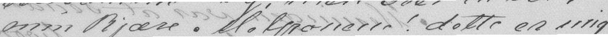min kjære Melponene! dette er mig
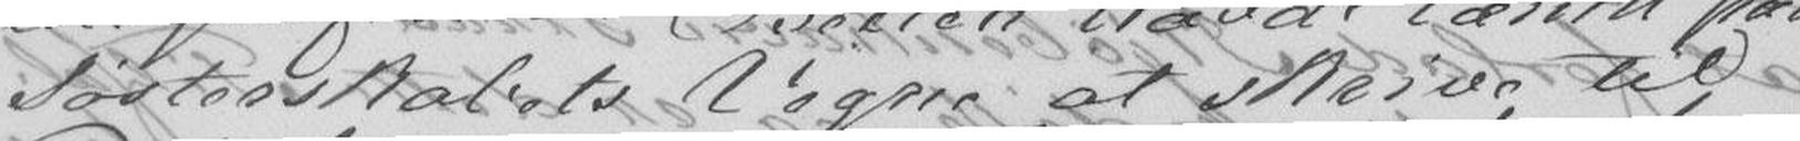Søsterskabets Vegne at skrive til
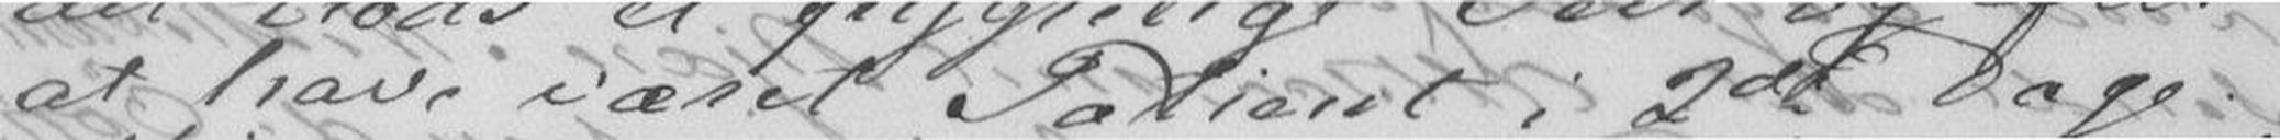at have været Patient i 2d Dage.
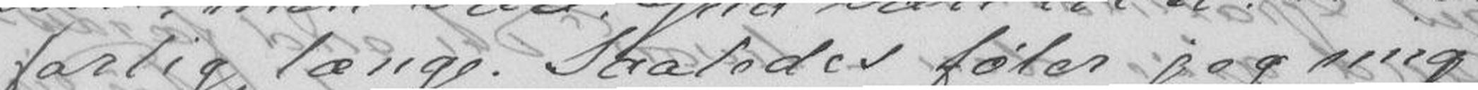farlig længe. Saaledes føler jeg mig
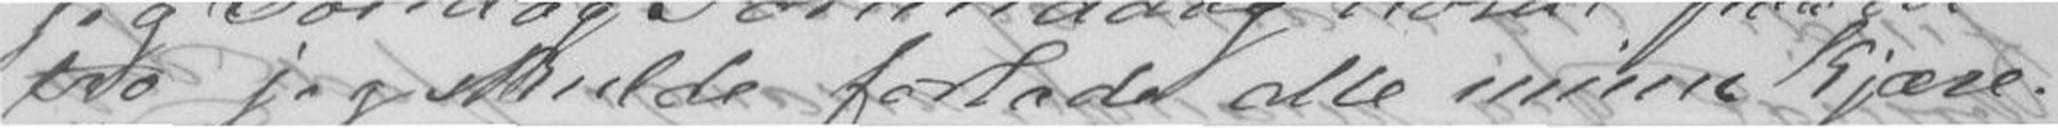tro jeg skulde forlade alle mine kjære.
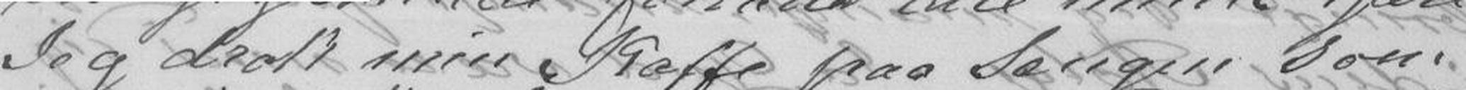Jeg drak min Kaffe paa Sengen som
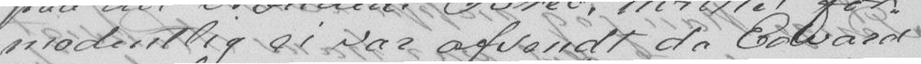modentlig ei var afsendt da Edvard
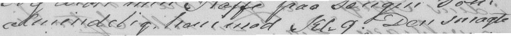almindelig, henimod Kl.9. Den smagte
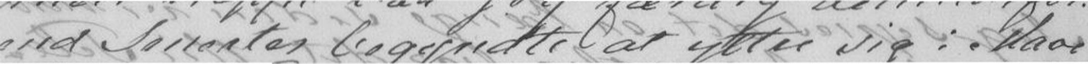end Smerter begyndte at yttre sig i Mave
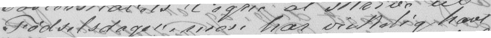Fødselsdagen, men har virkelig havt
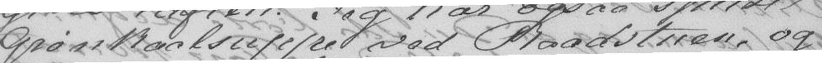Grønkaalsuppe ved Raadstuen, og
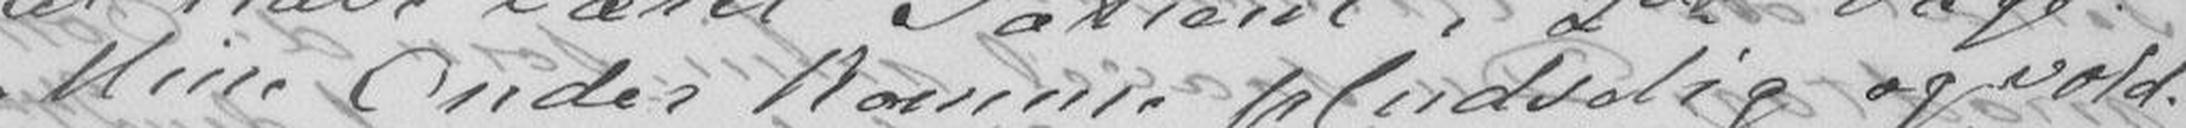Mine Onder kommer pludselig og vild-
